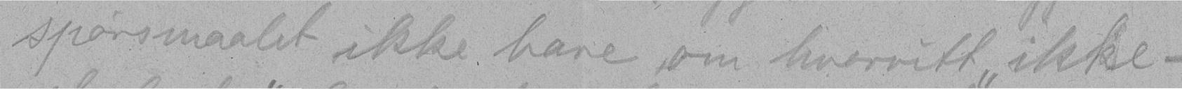spørsmaalet ikke bare om hvorvidt "ikke-
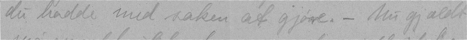du hadde med saken at gjøre. - Nu gjaldt
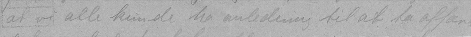at vi alle kunde ha anledning til at ta affære.
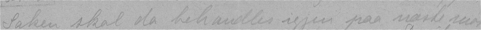Saken skal da behandles igjen paa næste møte.
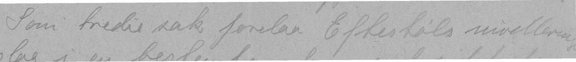Som tredie sak forelaa Eftestøls nivellerings-
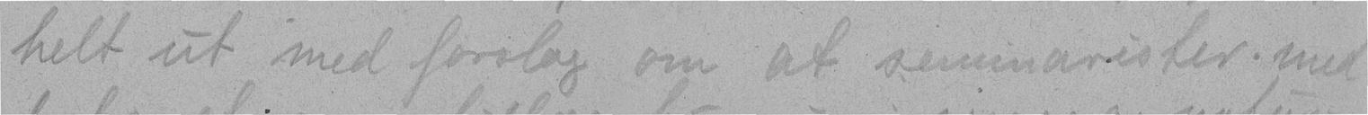helt ut med forslag om at seminarister med
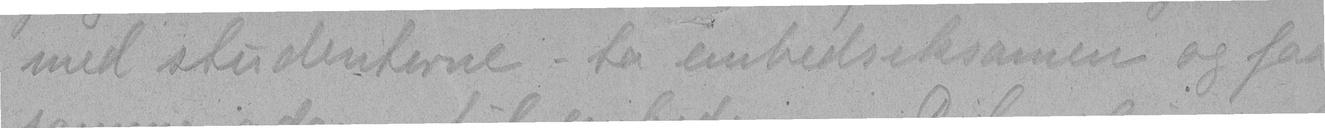med studenterne, ta embedseksamen og faa
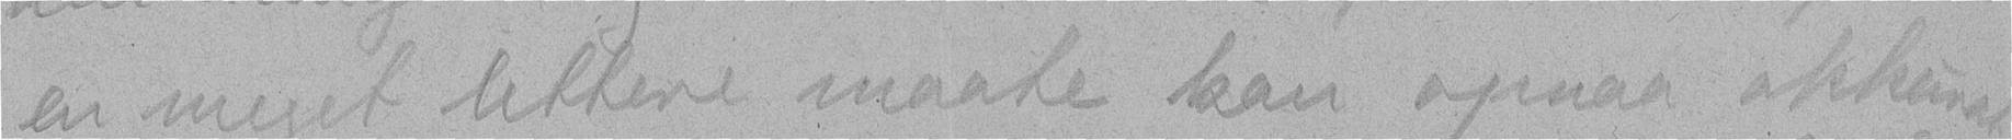en meget lettere maate kan opnaa akkurat
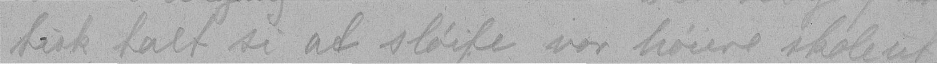tisk talt si at sløife vor høiere skoleut-
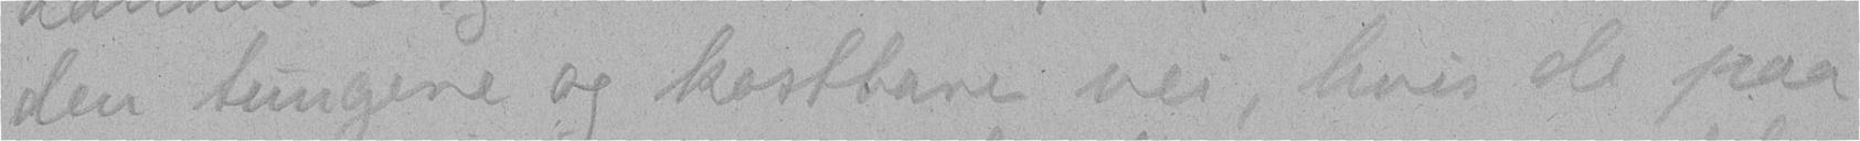den tungere og kostbare vei, hvis de paa
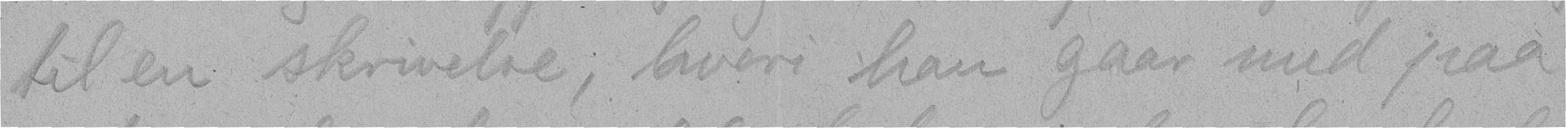til en skrivelse, hvori han gaar med paa
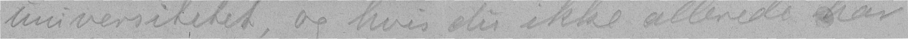universitetet, og hvis du ikke allerede har
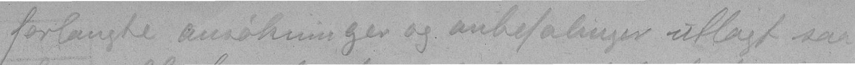forlangte ansøkninger og anbefalinger utlagt saa
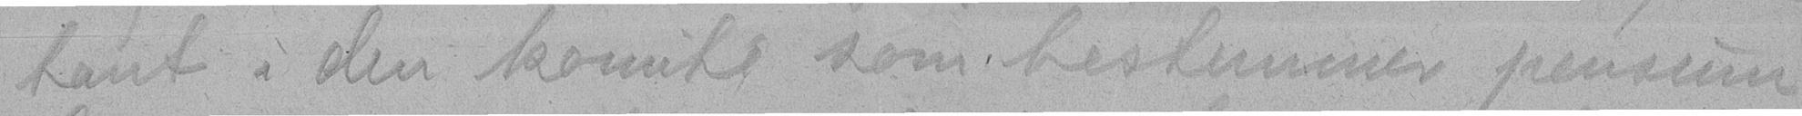tant i den komite som bestemmer pensum
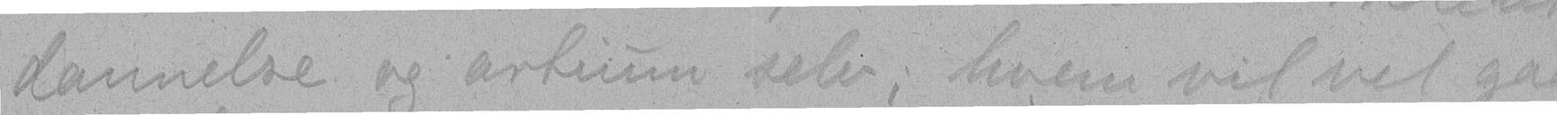dannelse og artium selv, hvem vil vel gaa
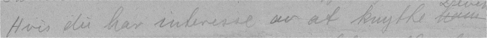Hvis du har interesse av at knytte ham
